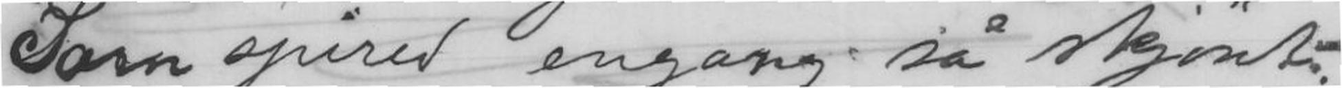Som spirer engang så skjønt.
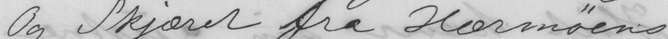Og Skjæret fra Hærmøens
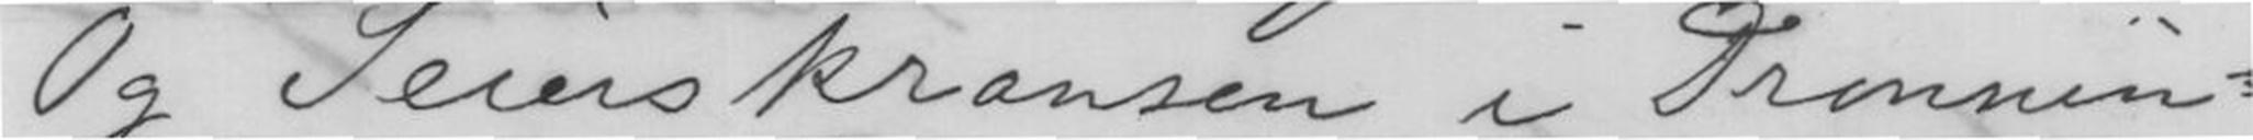Og Seierskransen i Dronnin-
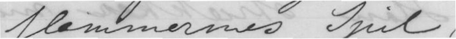flammermes Spil,
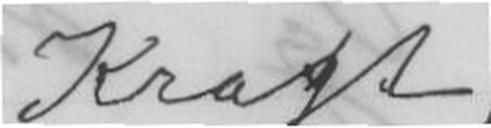Kraft,
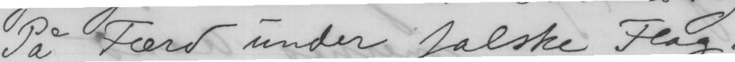På Færd under falske Flag.
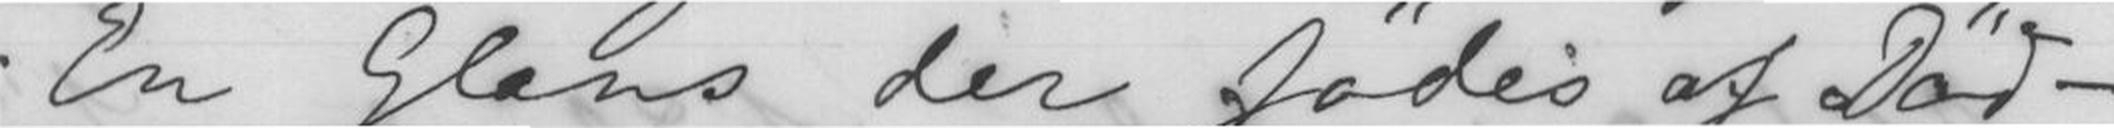- En Glands der fødes af Død -
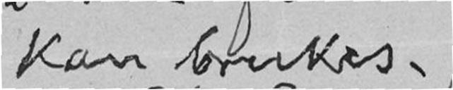kan brukes.
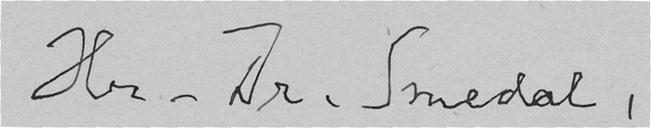Hr. Dr. Smedal,
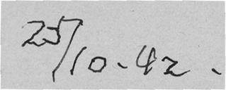25/10. 42.
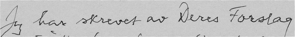Jeg har skrevet av Deres Forslag
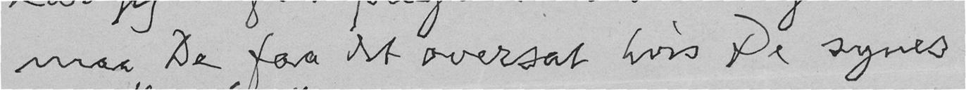maa De faa det oversat hvis De synes
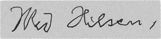Med Hilsen,
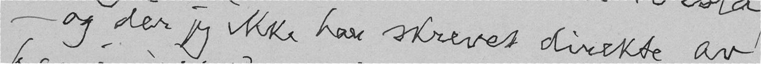- og der jeg ikke har skrevet direkte av
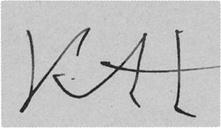KH
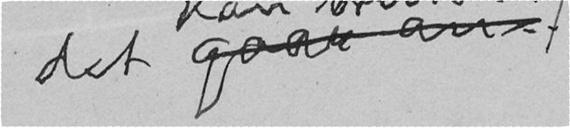det gaar an.
